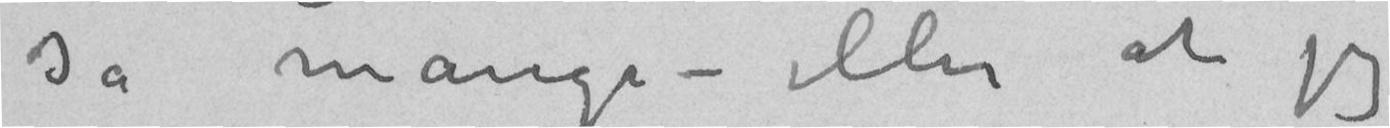så mange – eller at jeg
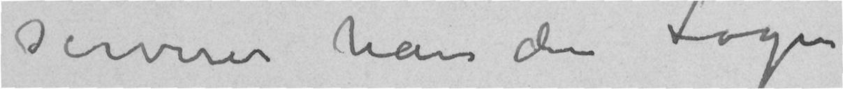serverer han den Løgn
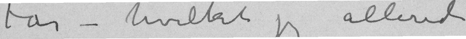Far – hvilket jeg allerede
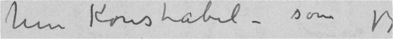hin Konstabel – som jeg
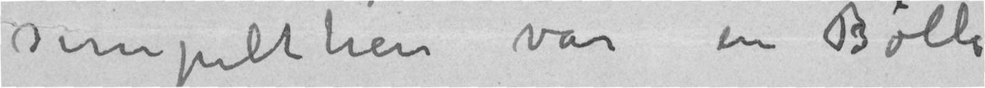simpelthen var en Bølle
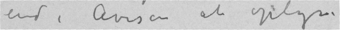end i Avisen at oplyse
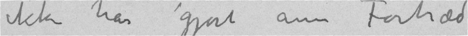ikke har gjort annen Fortræd
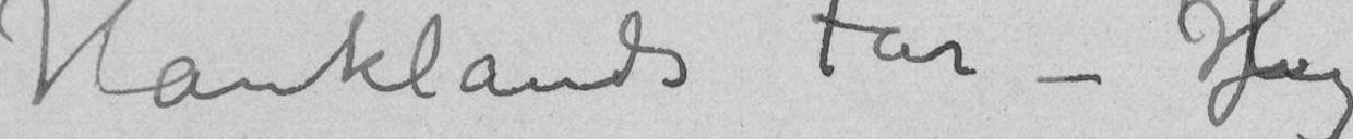Hauklands Far – Jeg
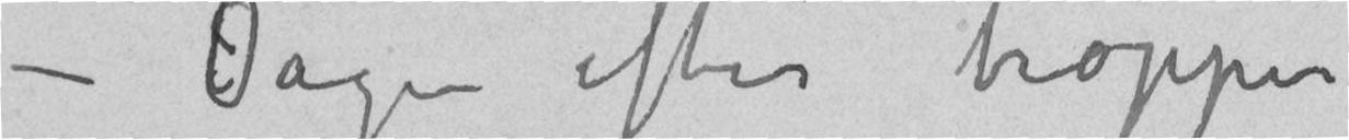– Dagen efter tropper
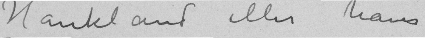Haukland eller hans
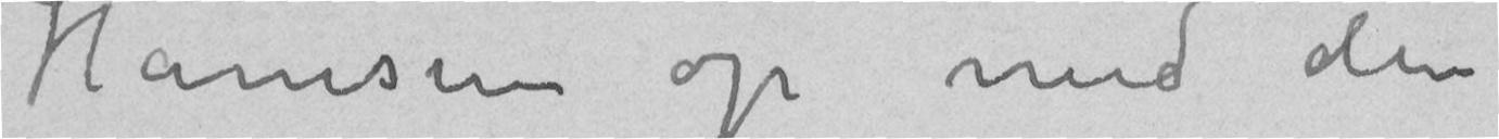Hamsun op med den
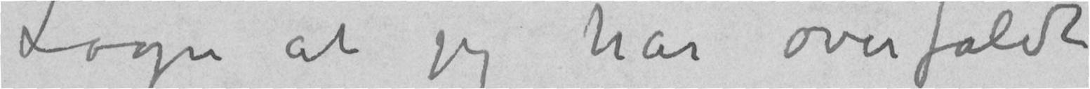Løgn at jeg har overfaldt
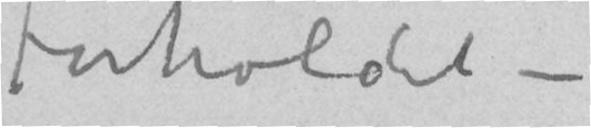Forholdet –
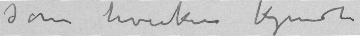som hverken kjendte
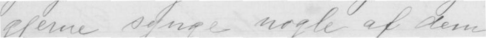gjerne synge nogle af dem
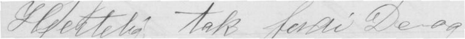Hjertelig tak for at De og
